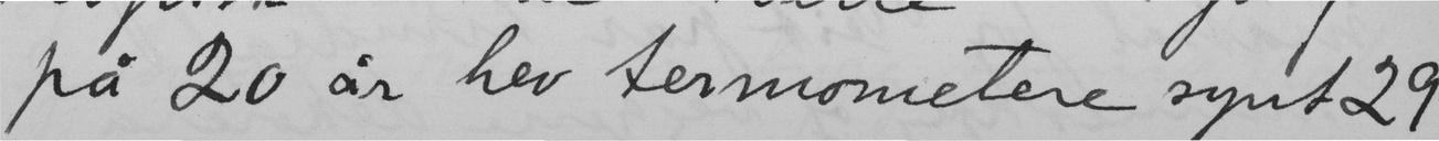på 20 år hev termometene synt 29
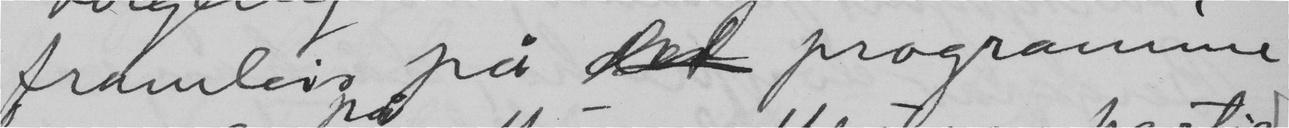framleis på det programme
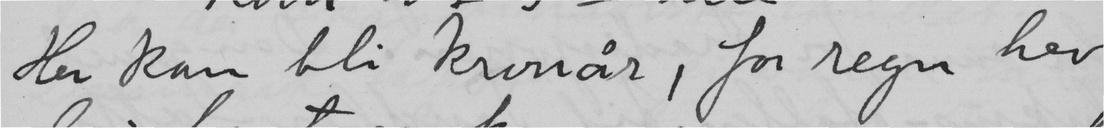Her kan bli kronår, for regn hev
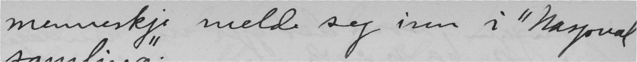menneskje melde seg inn i "Nasjonal
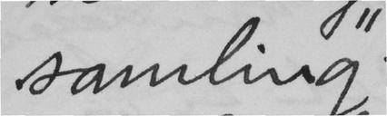samling".
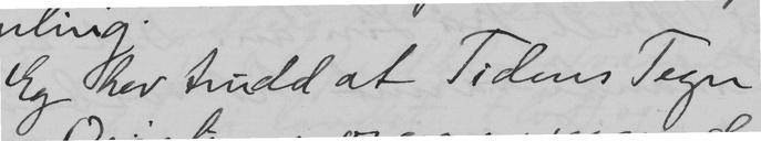Eg hev trudd at Tidens Tegn
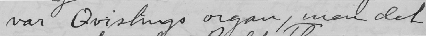var Qvislings organ, men det
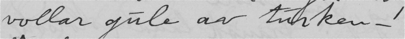vollar gule av turken –
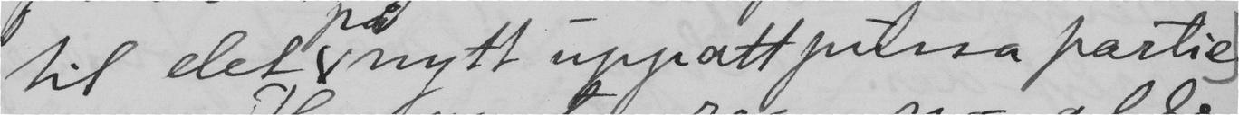til det pånytt uppattpussa partie.
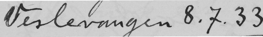Veslevangen 8.7.33
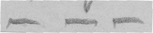- - -
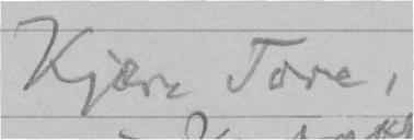Kjære Tore,
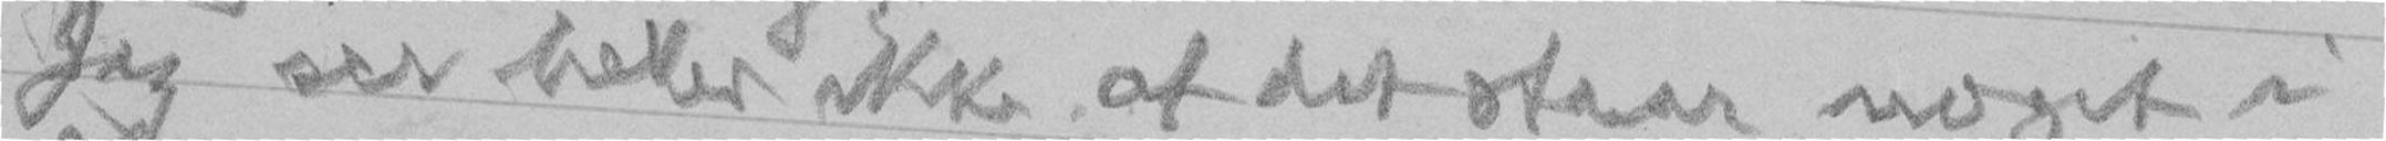Jeg ser heller ikke at det staar noget i
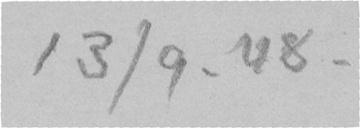13/9. 48.
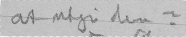at utgi den?
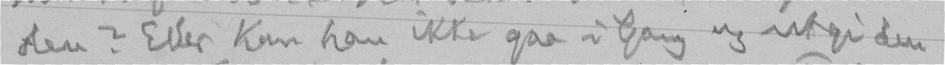den? Eller kan han ikke gaa i Gang og utgi den
